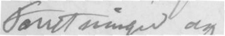Foretninger og
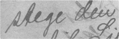stege den
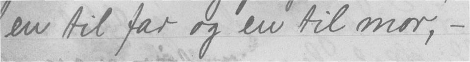en til far og en til mor, -
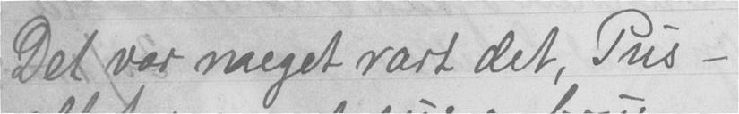Det var meget rart det, Pus -
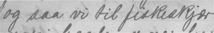og saa vi til fiskeskjær
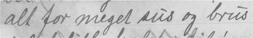alt for meget sus og brus
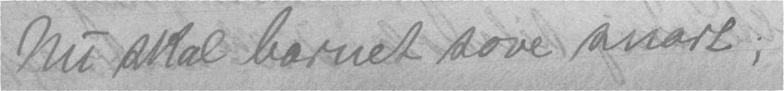nu skal barnet sove snart;
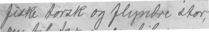fiske torsk og flyndre stor,
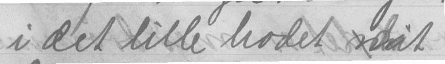i det lille hodet dit!
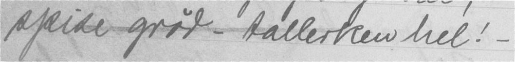spise grød - tallerken hel! -
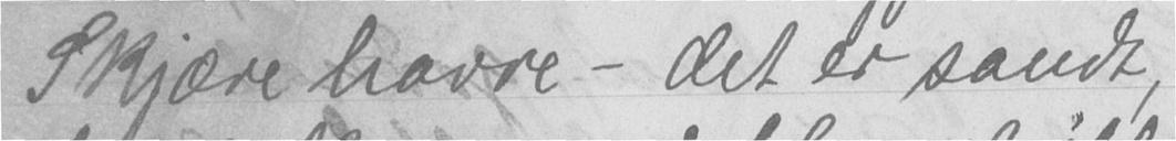Skjære havre - det er sandt,
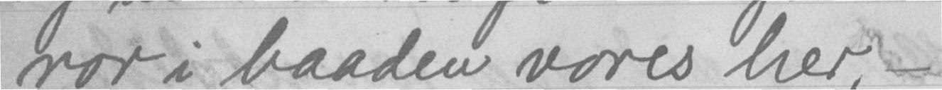ror i baaden væres her, -
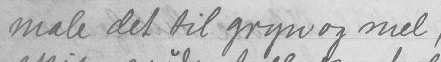male det til gryn og mel,
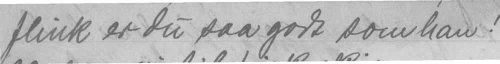flink er du saa godt som han!
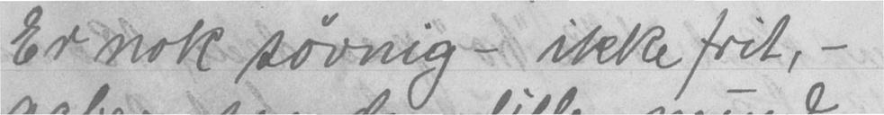Er nok søvnig - ikke frit, -
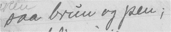saa brun og pen;
